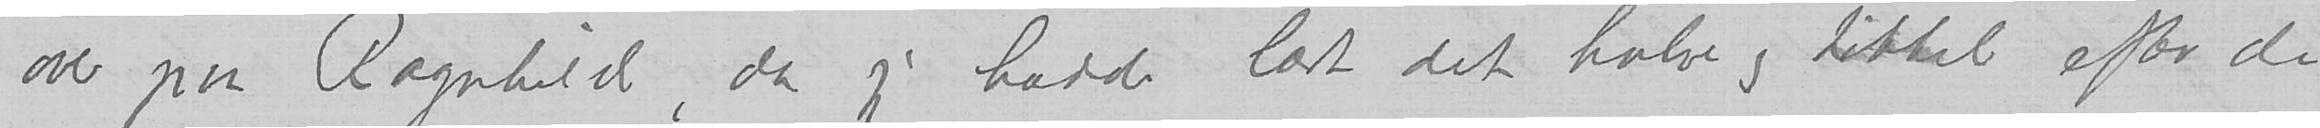over paa Ragnhild, da jeg hadde læst det halve og littil efter de
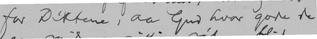for Diktene, aa Gud hvor gode de
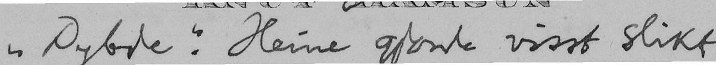"Dybde". Heine gjorde visst slikt
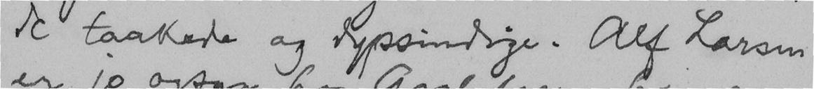de taakede og dypsindige. Alf Larsen
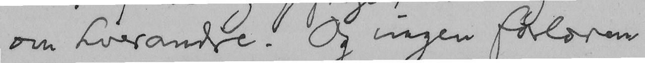om hverandre. Og ingen forloren
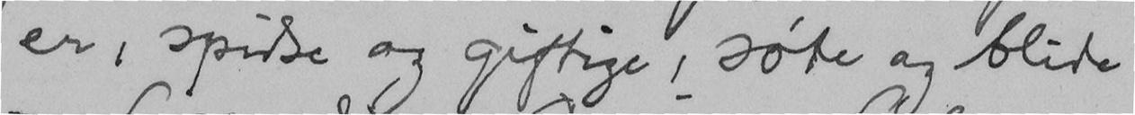er, spidse og giftige, søte og blide
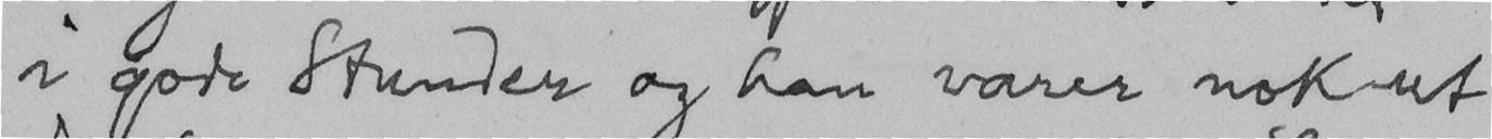i gode Stunder og han varer nok ut
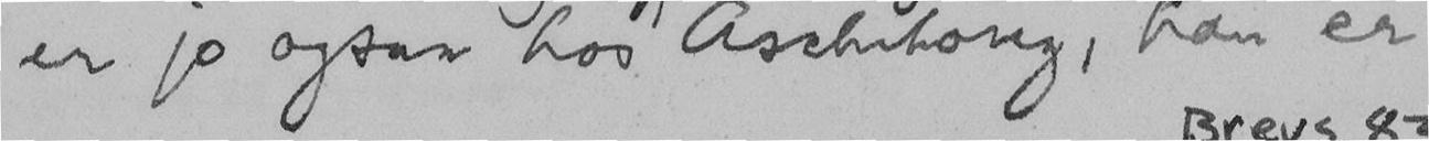er jo ogsaa hos Aschehoug, han er
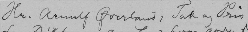Hr. Arnulf Øverland, Tak og Pris
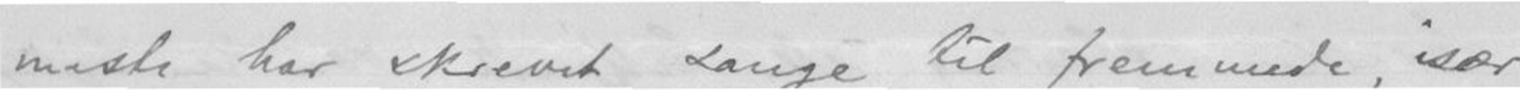mæste har skrevet sange til fremmede, især
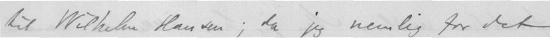til Wilhelm Hansen, da jeg nemlig for det
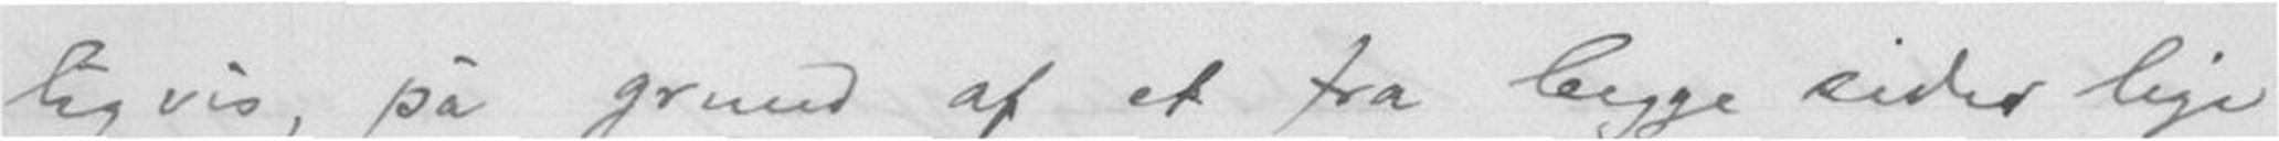ligvis, på grund af et fra begge sider lige
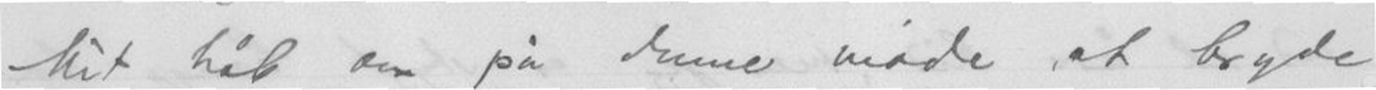Mit håb er på denne måde at bryde
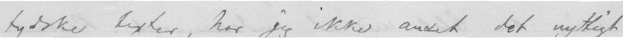tydske texter, har jeg ikke anset det nyttigt
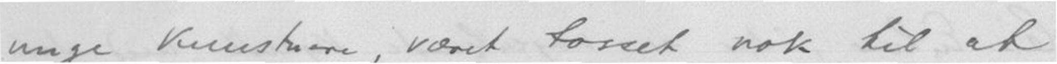unge kunstnere, vært tosset nok til at
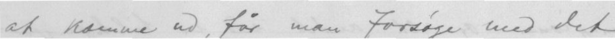at komme ud, får man forsøge med det
